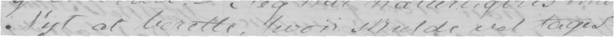Nyt at berette, hvori skulde vel tages
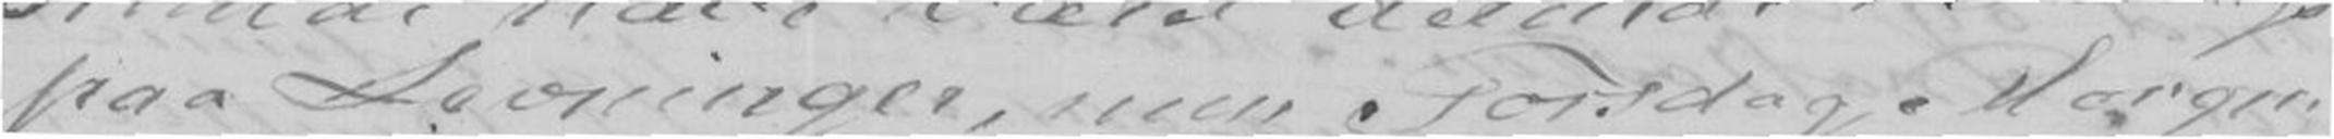paa Levninger, men Torsdag Morgen
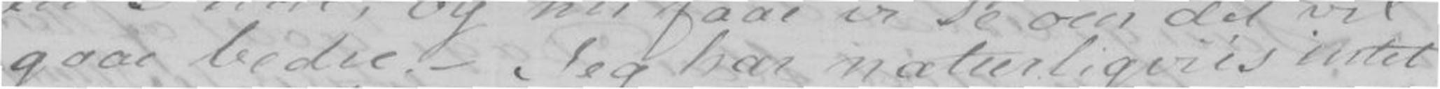gaae bedre. - Jeg har naturligviis intet
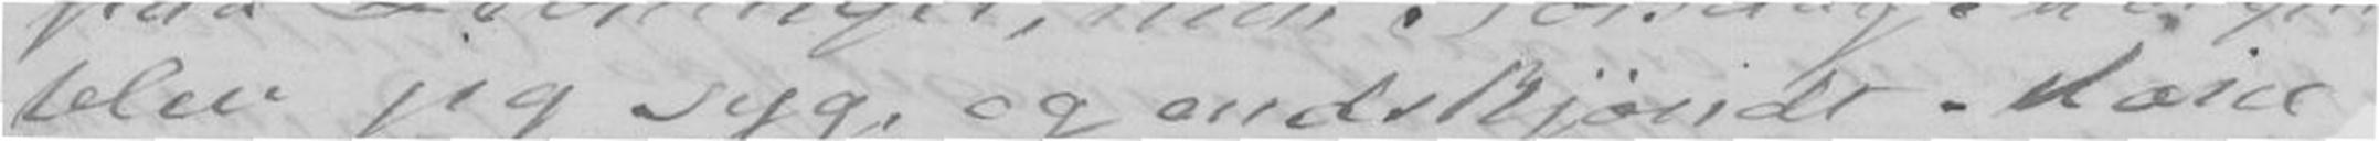blev jeg syg, og endskjøndt Marie
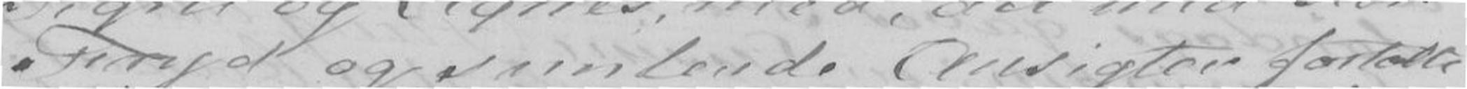Fryd og smilende Ansigter fortalte
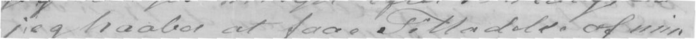jeg haaber at faae Tilladelse af min
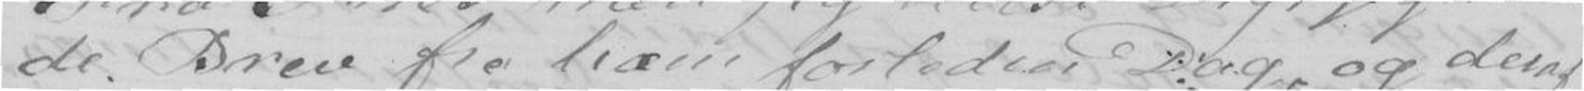de Brev fra ham forleden Dag, og deraf
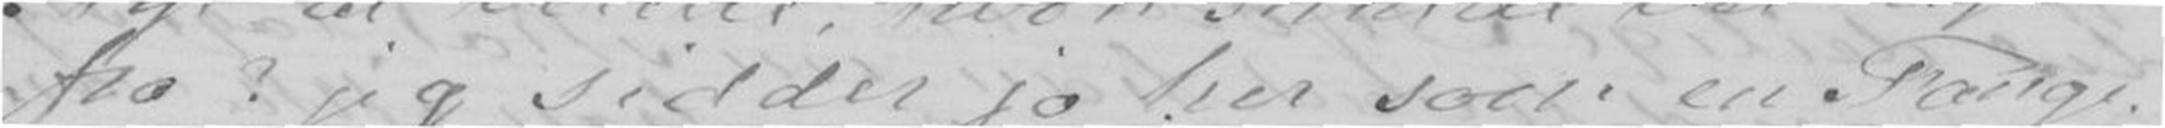fra? jeg sidder jo her som en Fange
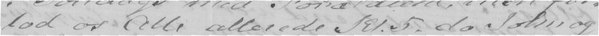lod os Alle allerede Kl.5. da John og
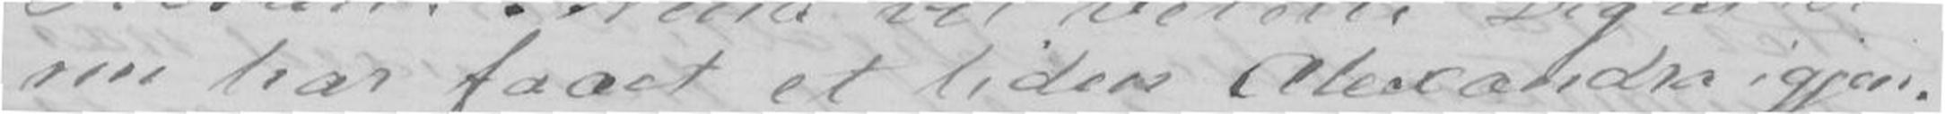nu har faaet et liden Alexandra igjen.
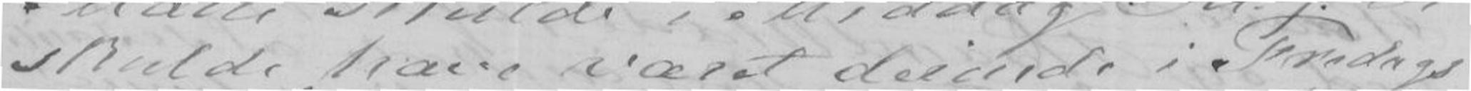skulde have været derinde i Fredags
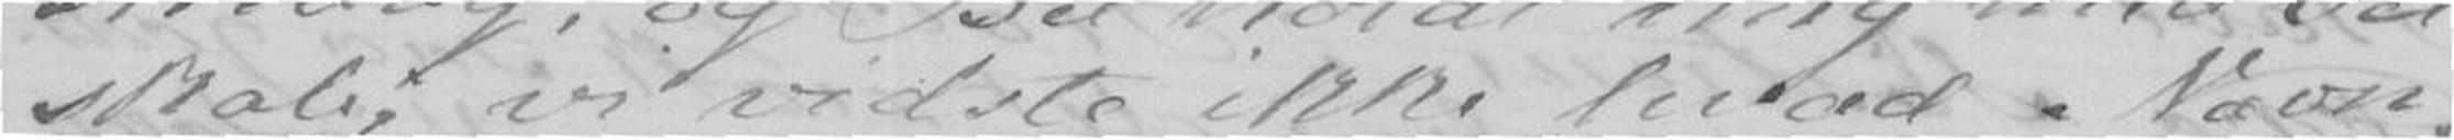skab; vi vidste ikke hvad Navn
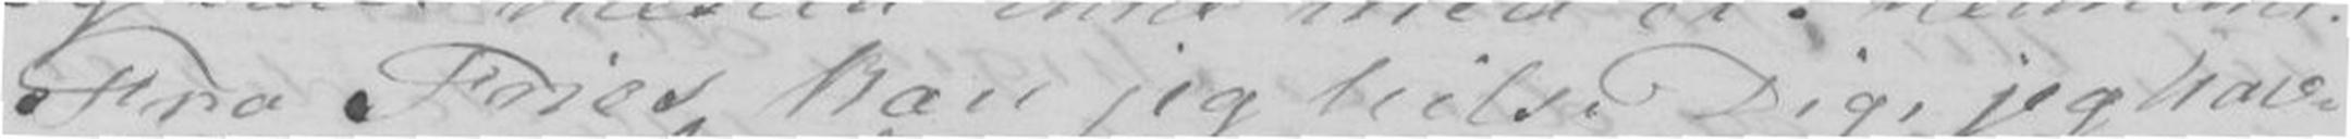Fra Fries kan jeg hilse Dig, jeg hav-
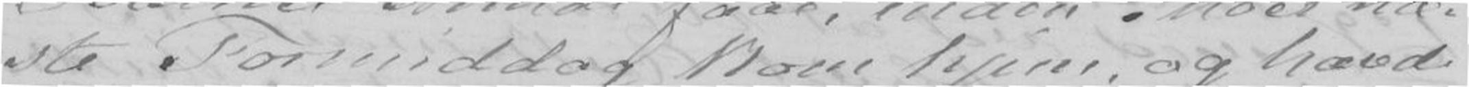ste Formiddag kom hjem, og havde
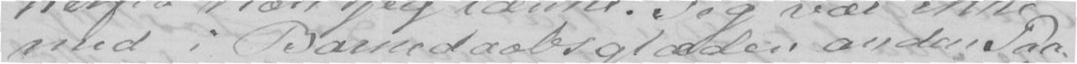med i Barnedaabsglæden anden Paa-
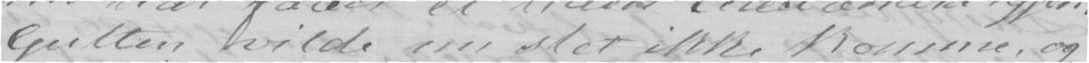Gutten vilde nu slet ikke komme, og
